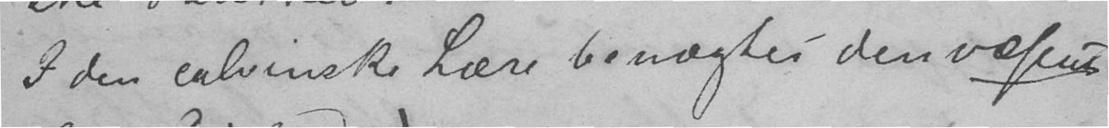I den calvinske Lære benægtes den væsent-
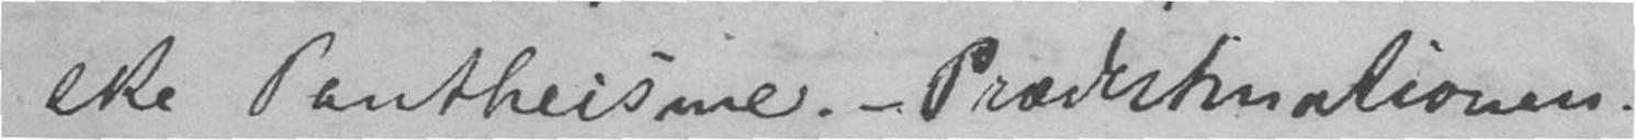ske Pantheisme. – Prædestationen.
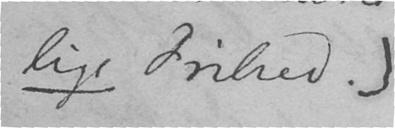lige Frihed.)
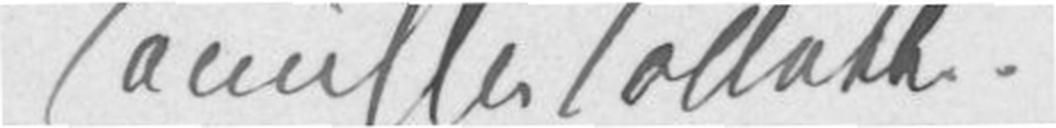Camilla Collett.
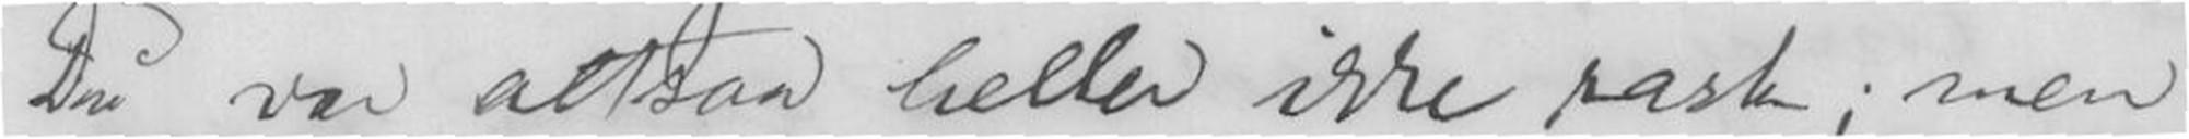Du var altsaa heller ikke rask; men
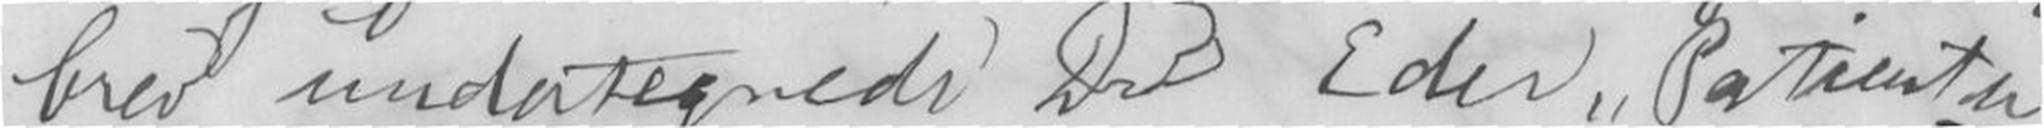brev undertegnede Du Eder Patienter;
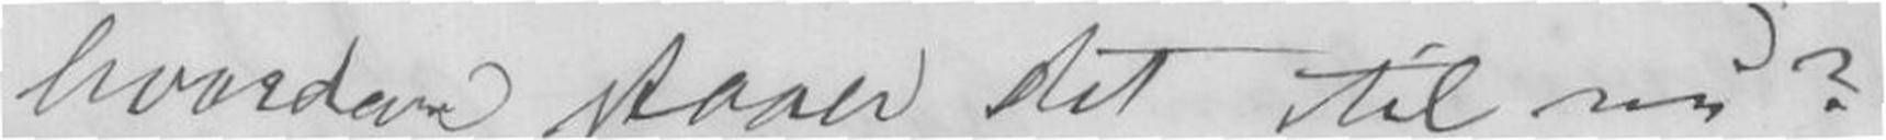hvordan staaer det til nu?
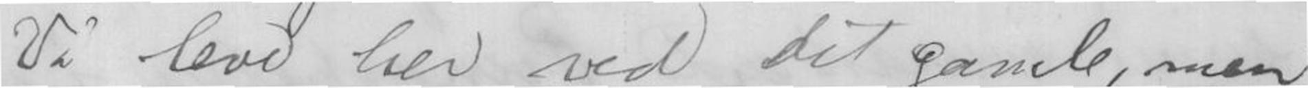Vi leve her ved det gamle, men
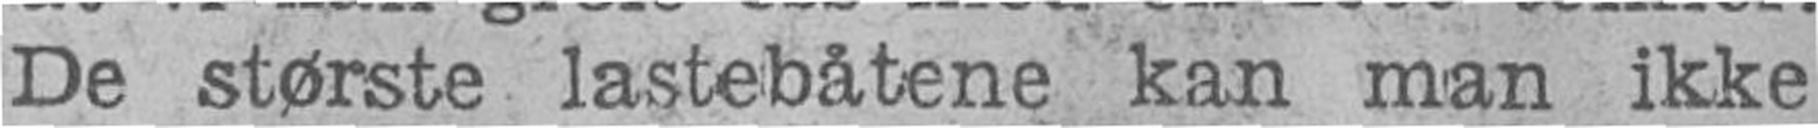De største lastebåtene kan man ikke
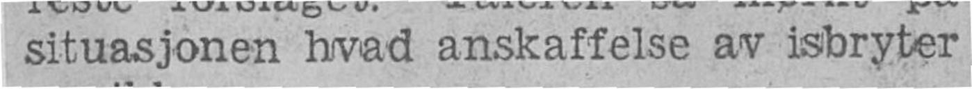situasjonen hvad anskaffelse av isbryter
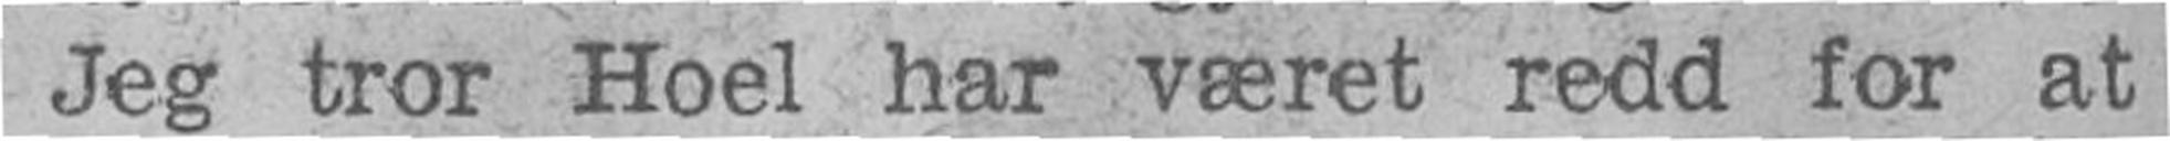Jeg tror Hoel har været redd for at
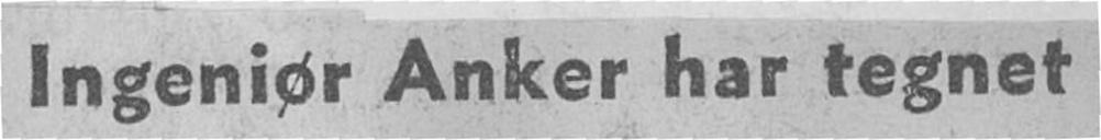Ingeniør Anker har tegnet
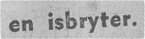en isbryter.
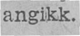angikk.
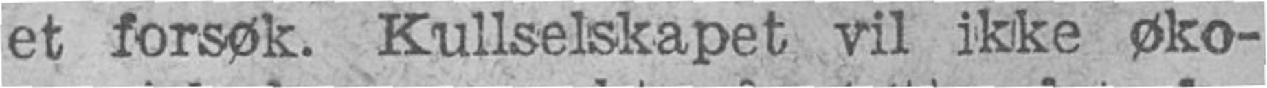et forsøk. Kullselskapet vil ikke øko-
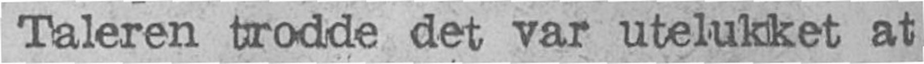Taleren trodde det var utelukket at
